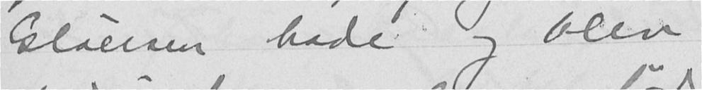Gløersen bade og blev
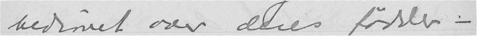bedrøvet over deres fødsler -
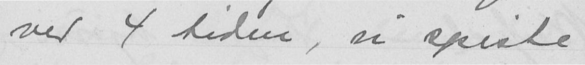ved 4 tiden, vi spiste
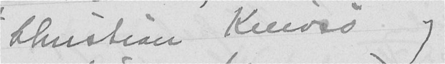Christian Knivsø og
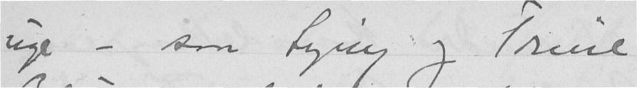uge - saa Signy og Trine
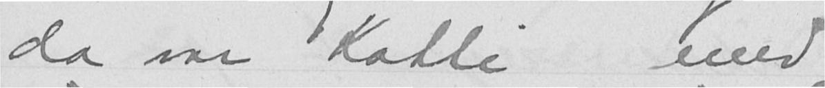da var Katti med
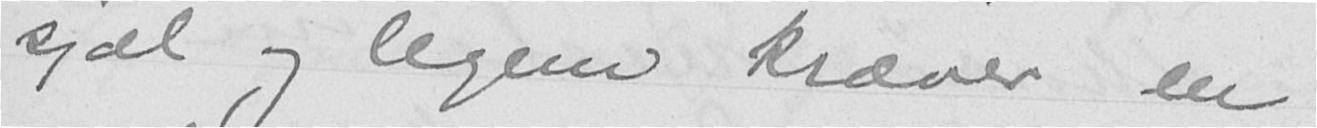sjæl og legem kræver en
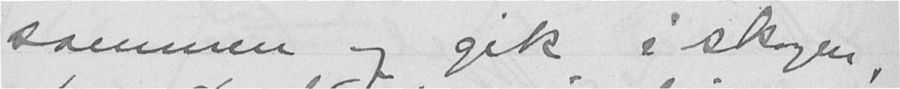sammen og gik i skogen,
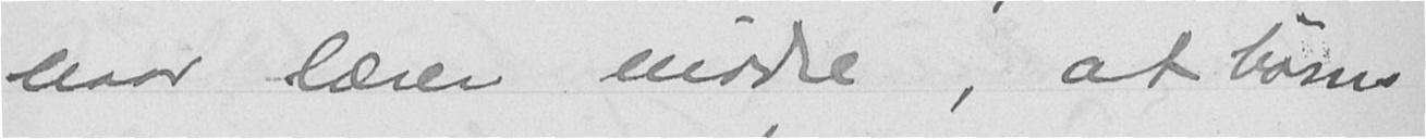naar lærer mødre, at børns
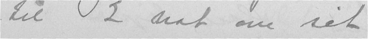til 2 nat om sit
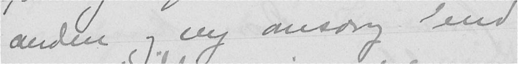anden og ny omsorg end
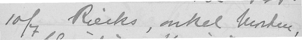10/7 Riecks, onkel Morten,
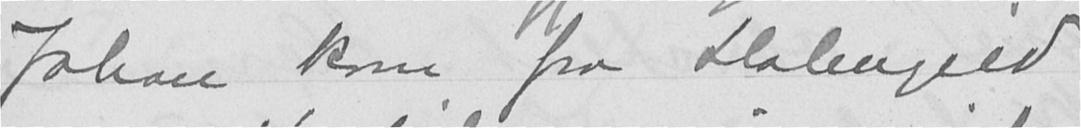Johan kom fra Holmgild
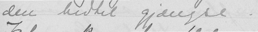den hidtil gjængse.
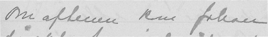Om aftenen kom Johan
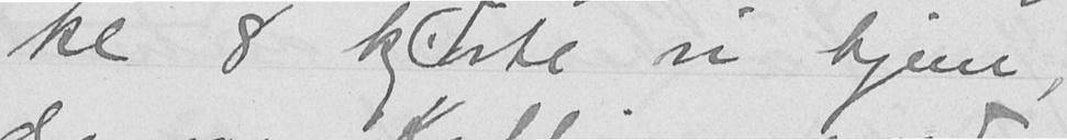kl 8 kjørte vi hjem,
